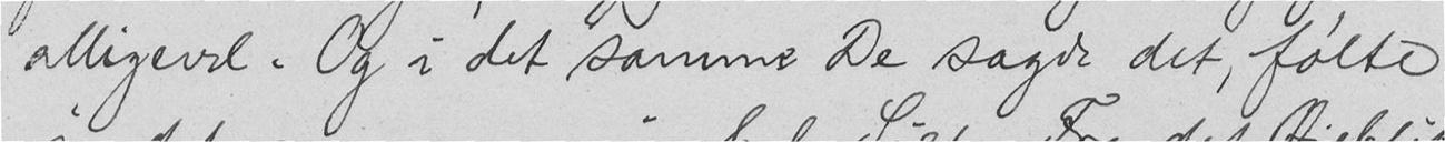alligevel. Og i det samme De sagde det, følte
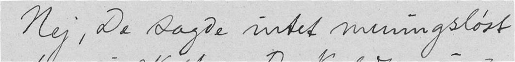Nei, De sagde intet meningsløst
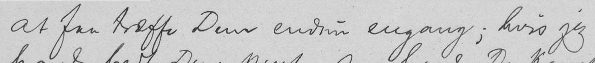at faa træffe Dem endnu engang; hvis jeg
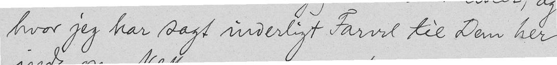hvor jeg har sagt inderligt Farvel til Dem her
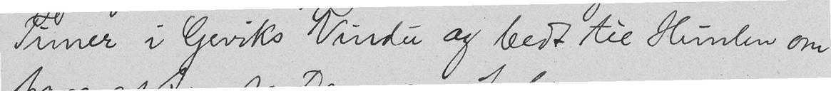Timer i Geviks Vindu og bedt til Himlen om
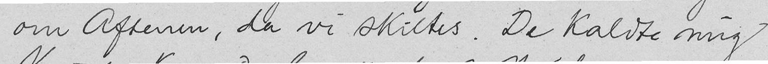om Aftenen, da vi skiltes. De kaldte mig
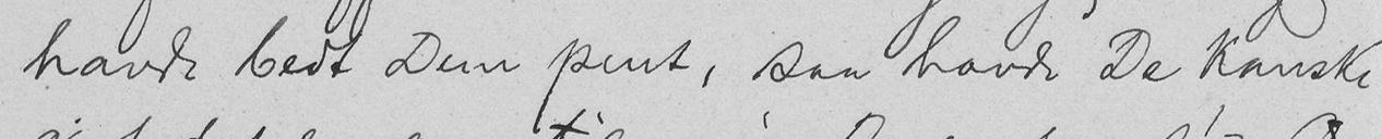havde bedt Dem pent, saa havde De kanske
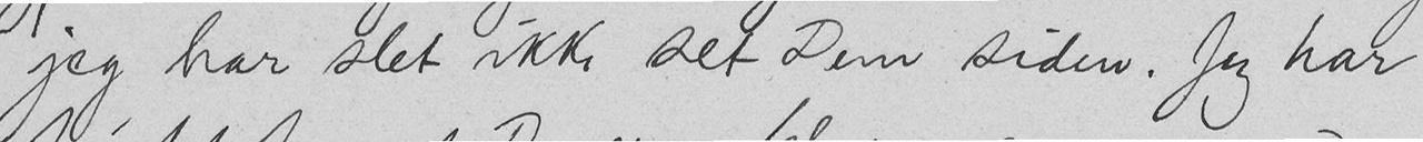jeg har slet ikke set Dem siden. Jeg har
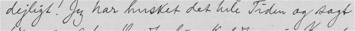dejligt! Jeg har husket det hele Tiden og sagt
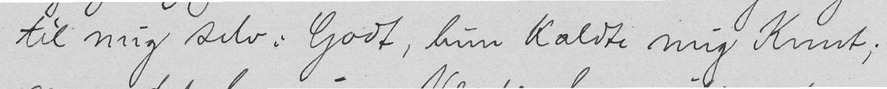til mig selv: Godt, hun kaldte mig Knut;
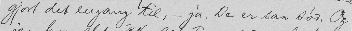gjort det engang til, - ja, De er saa sød. Og
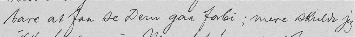bare at faa se Dem gaa forbi; mere skulde jeg
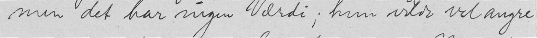men det har ingen Værdi; hun vilde vel angre
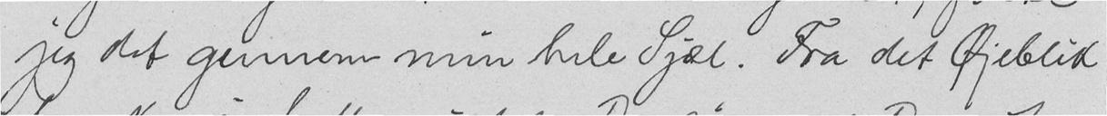jeg det gennem min hele Sjæl. Fra det Øjeblik
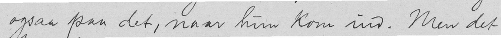ogsaa paa det, naar hun kom ind. Men det
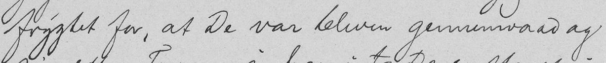tryglet for, at De var bleven gennemvaad og
